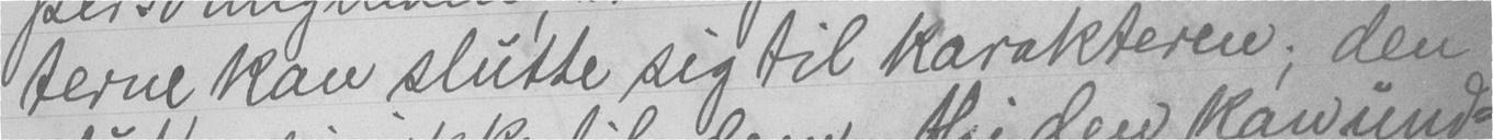terne kan slutte sig til karakteren; den
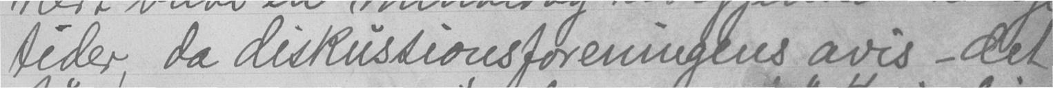tider, da diskussionsforeningens avis - det
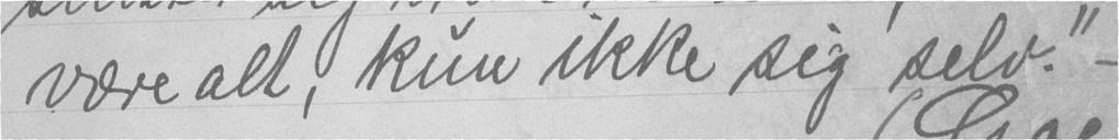være alt, kun ikke sig selv." -
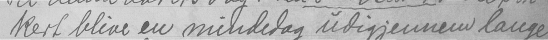kert blive en mindedag udigjennem lange
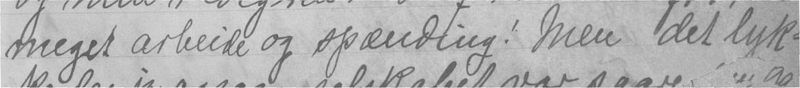meget arbeide og spænding! Men det lyk-
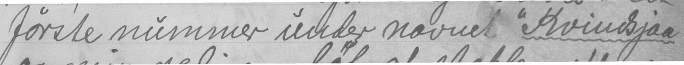første nummer under navnet "Kvindsjaa"
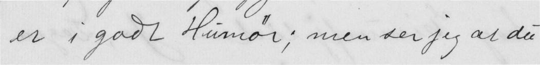er i godt Humør; men ser jeg at du
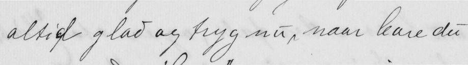altid glad og tryg nu, naar bare du
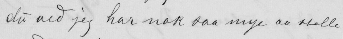du ved jeg har nok saa nye aa stelle
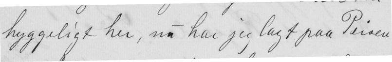hyggeligt her, nu har ger lagt paa Peisen
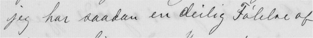jeg har saadan en derlig Følelse af
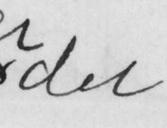det
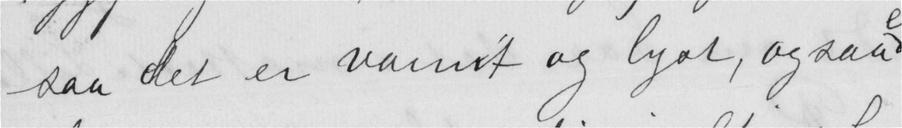saa det er vannet og lyst, ogsaa
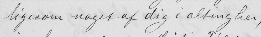ligesom noget af dig i alting her,
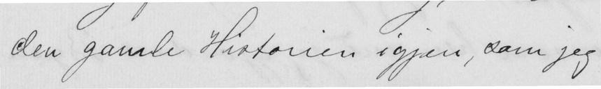den gamle Historien igjen, som jeg
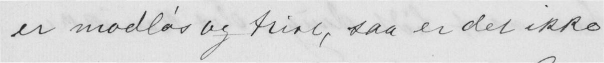er modløs og trist, saa er det ikke
